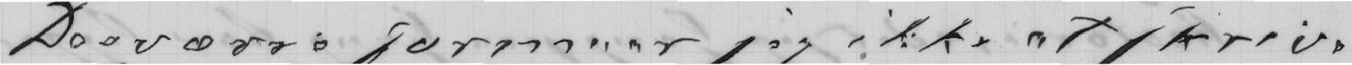Desværre formaar jeg ikke at skrive
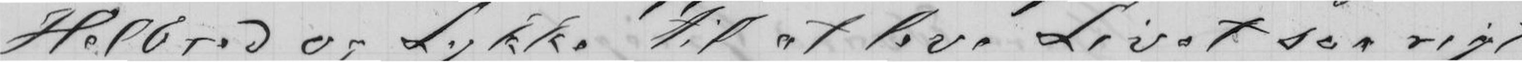Helbred og Lykke til at leve Livet saa rigt
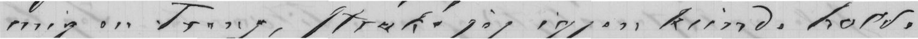mig en Trang, straks jeg igjen kunde holde
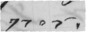ner.
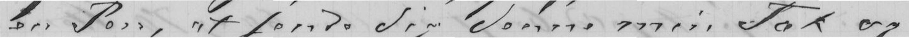en Pen, at sende dig denne min Tak og
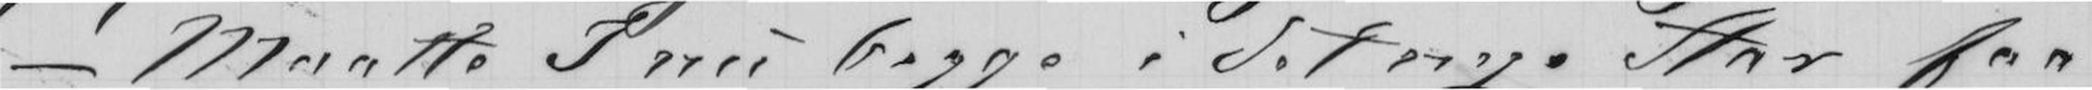- Maatte I nu begge i det nye Aar faa
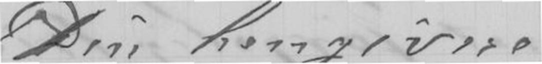Din hengivne
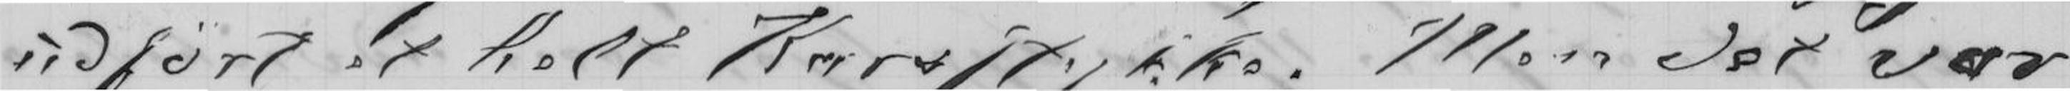udført et helt Karstykke. Men det var
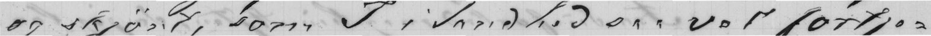og skjønt, som I i Sandhed saa vel fortje-
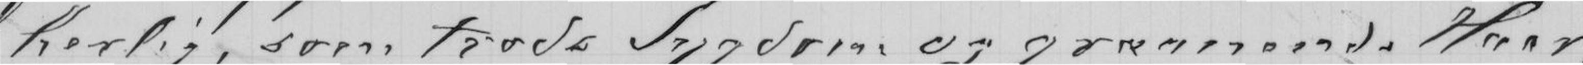herlig, som trods Sygdom og graanende Haar,
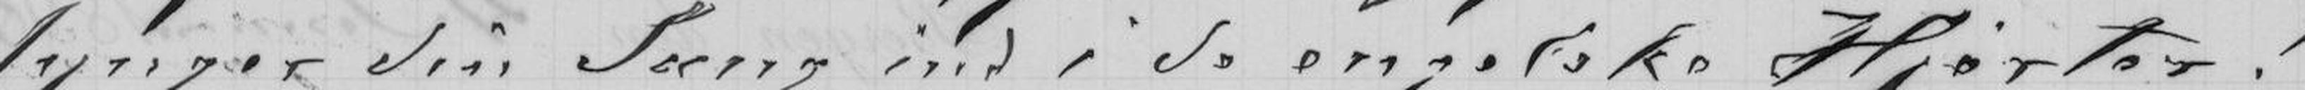synger din Sang ind i de engelske Hjerter!
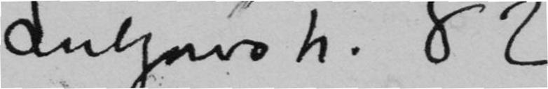Lützowstr. 82
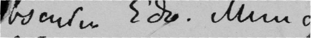bsender Edv. Munc
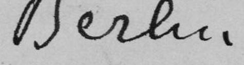Berlin
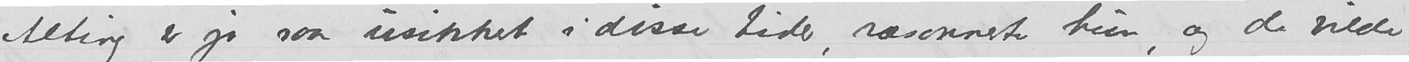Alting er jo saa usikkert i disse tider, ræsonnerte hun, og de vilde
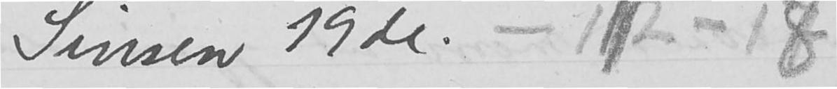Sinsen 19de
